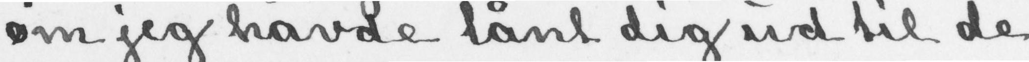om jeg havde lånt dig ud til de
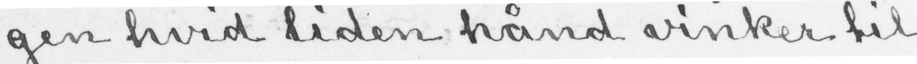gen hvid liden hånd vinker til
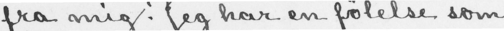fra mig! Jeg har en følelse som
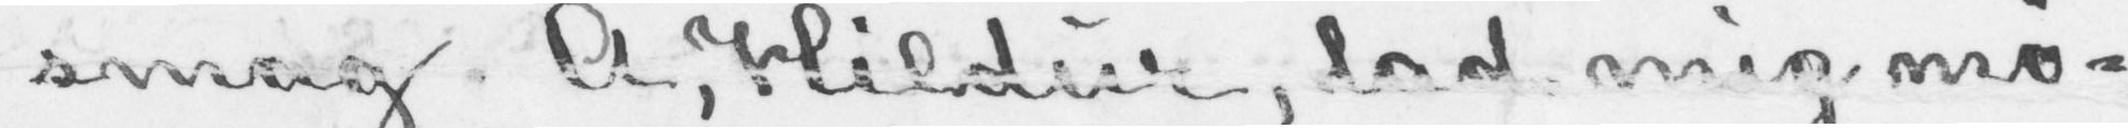smag. Å, Hildur, lad mig mø-
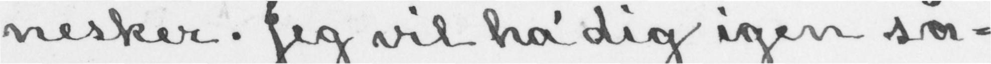nesker. Jeg vil ha' dig igen så-
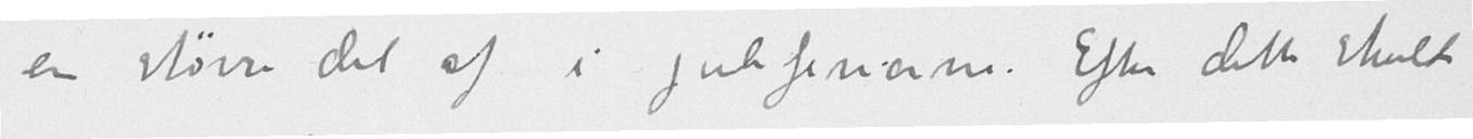en større del af i juleferierne. Efter dette skulde
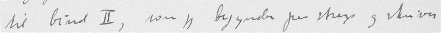til bind II, som jeg begynder paa strax og skriver
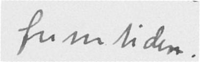fremtiden.
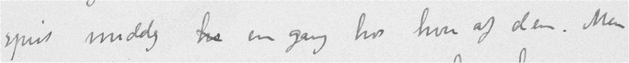spist middag hos en gang hos hver af dem. Men
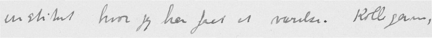institut hvor jeg har faat et værelse. Kollegerne,
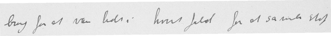brug for at være lidt i hvert fald for at samle stof
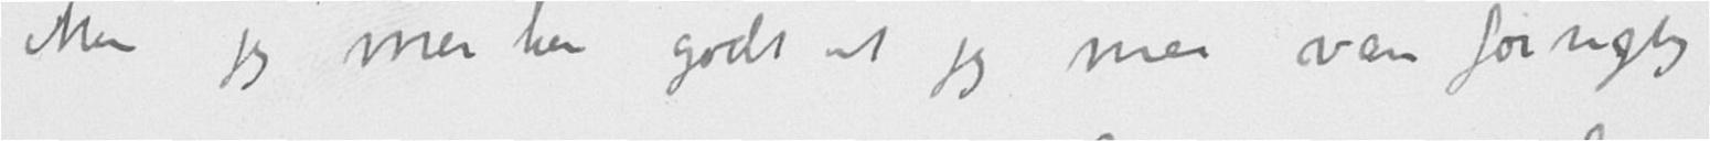Men jeg merker godt at jeg maa være forsigtig
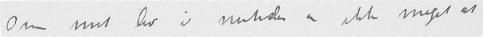Om mit liv i nutiden er ikke meget at
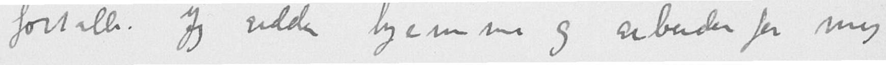fortælle. Jeg sidder hjemme og arbeider for mig
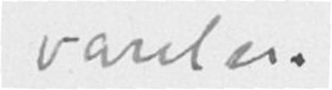værelse.
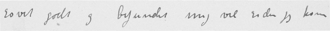sovet godt og befundet mig vel siden jeg kom
